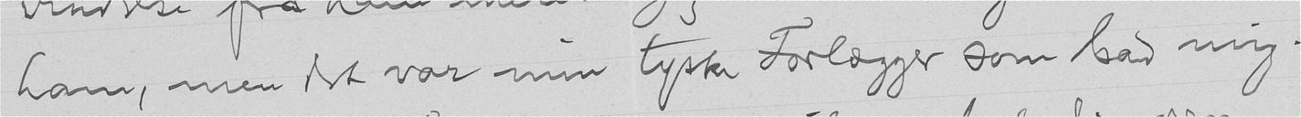ham, men det var min tyske Forlægger som bad mig.
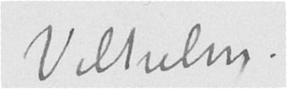Vilhelm.
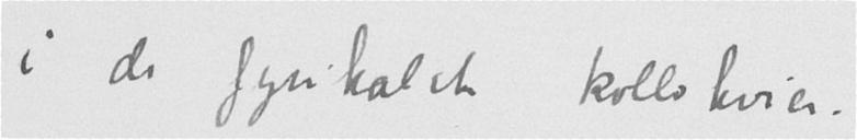i de fysikalske kollokvier.
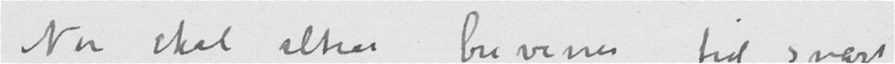Nu skal altsaa brevenes tid snart
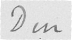Din
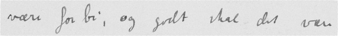være forbi, og godt skal det være
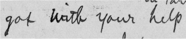got with your help
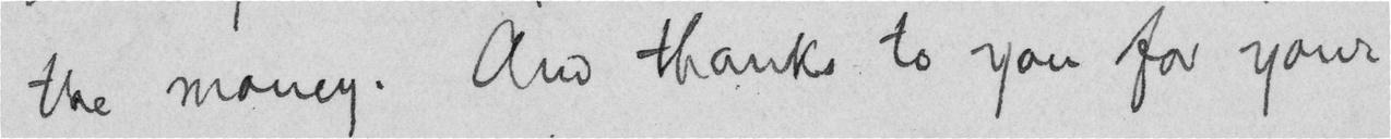the money. And thanks to you for your
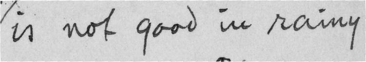is not good in rainy
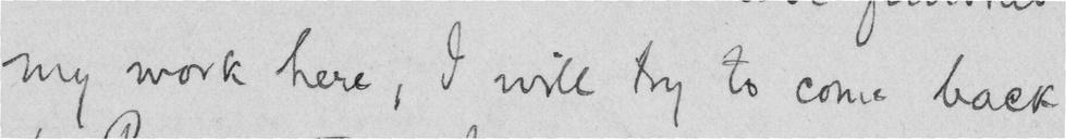my work here, I will try to come back
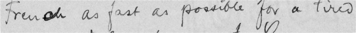French as fast as possible for a tired
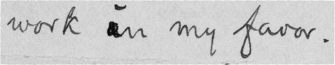work in my favor.
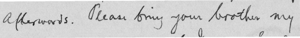afterwords. Please bring your brother my
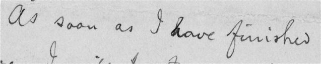As soon as I have finished
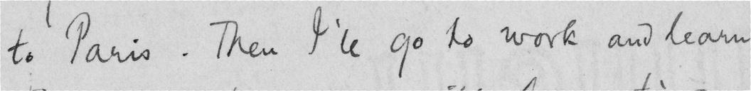to Paris. Then I'll go to work and learn
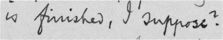is finished, I suppose?
